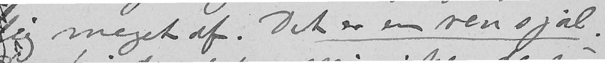lig meget af. Det er en ren sjæl.
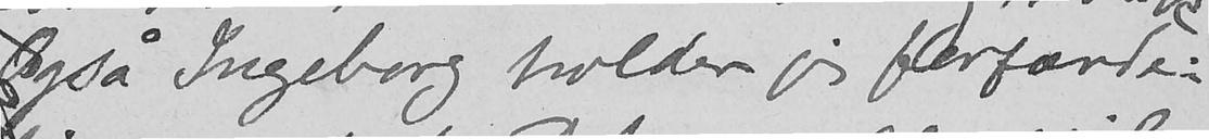Også Ingeborg holder jeg forfærde-
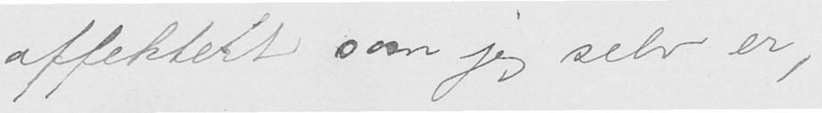affektert som jeg selv er,
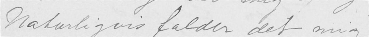Naturligvis falder det mig
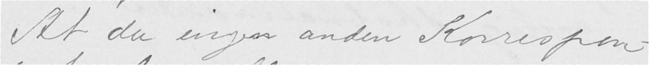At du ingen anden Korrespon-
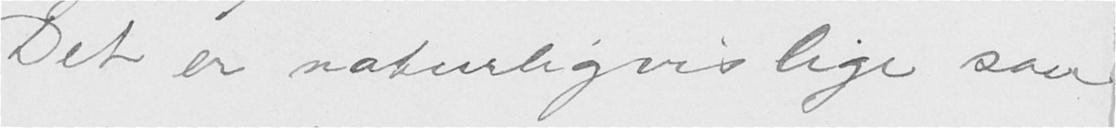Det er naturligvis lige saa
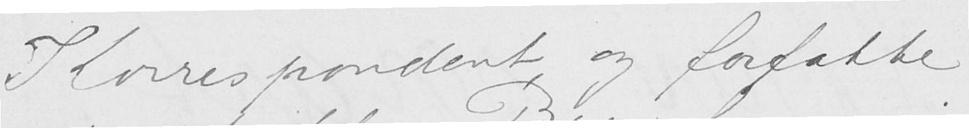Korrespondent, og forfatte
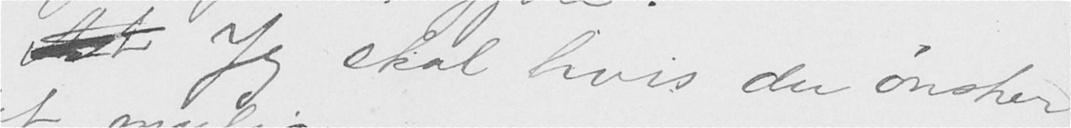Hr Jeg skal hvis du ønsker
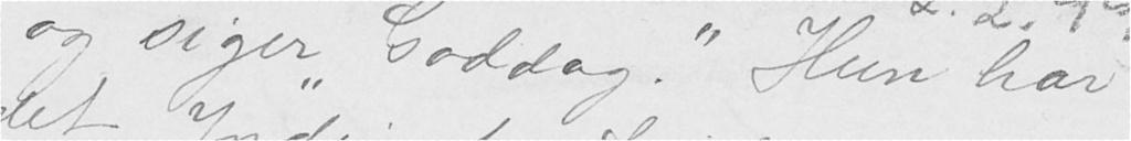og siger "Goddag". Hun har
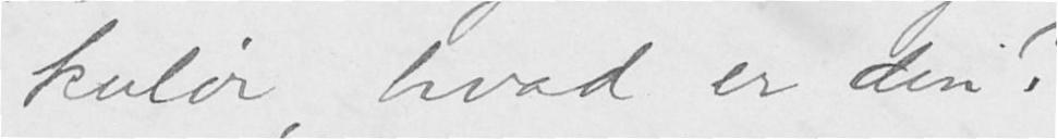kulør, hvad er din?
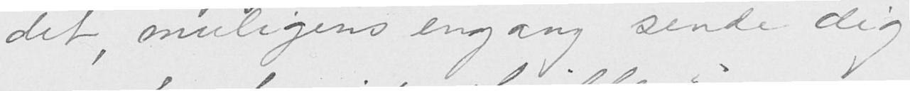det, muligens engang sende dig
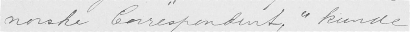norske Correspondent," kunde
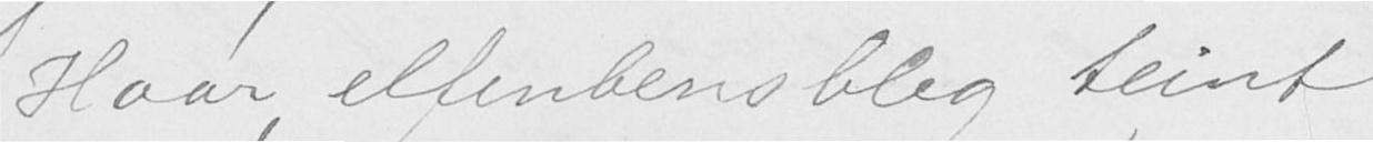Haar, elfenbensblegt teint
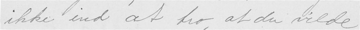ikke ind at tro, at du vilde
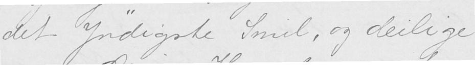det yndigste Smil, og deilige
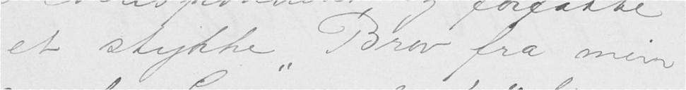et stykke "Brev fra min
